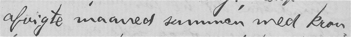afvigte maaned sammen med kron-
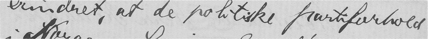erindret, at de politiske partiforhold
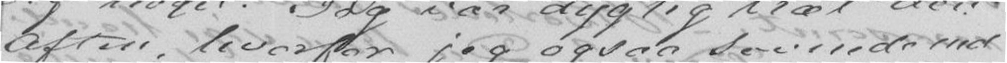Aften, hvorfor jeg ogsaa sovnede ind
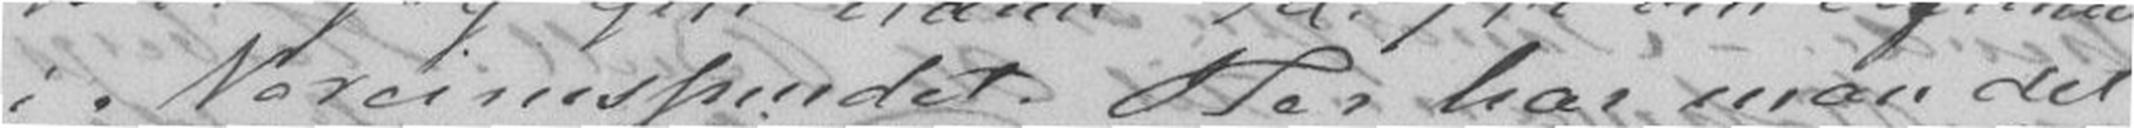i Noreimssundet. Her har man det
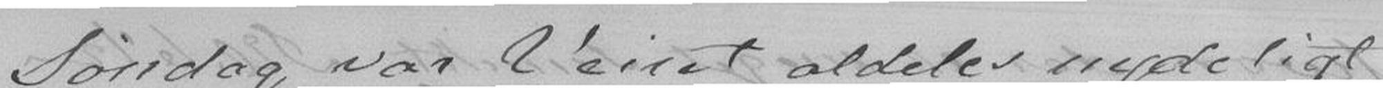Søndag var Veiret aldeles nydeligt
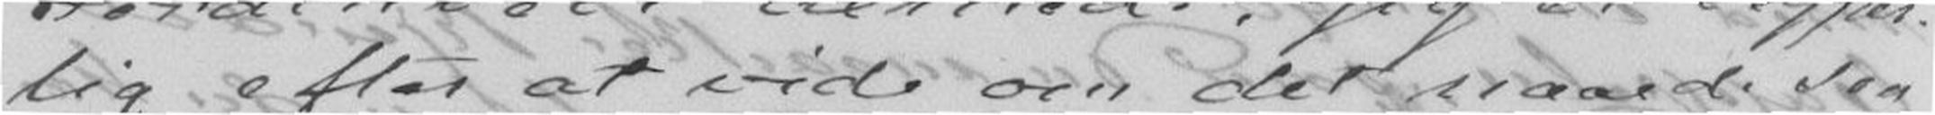lig efter at vide om det naaede saa
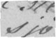sjø
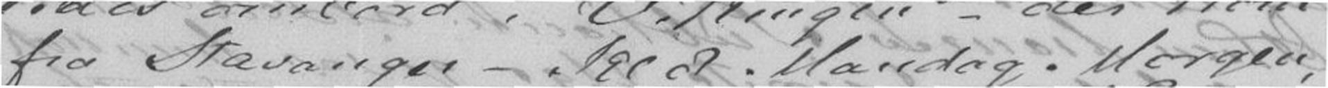fra Stavanger - Kl 8 Mandag Morgen,
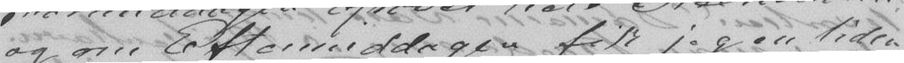og om Eftermiddagen fik jeg en liden
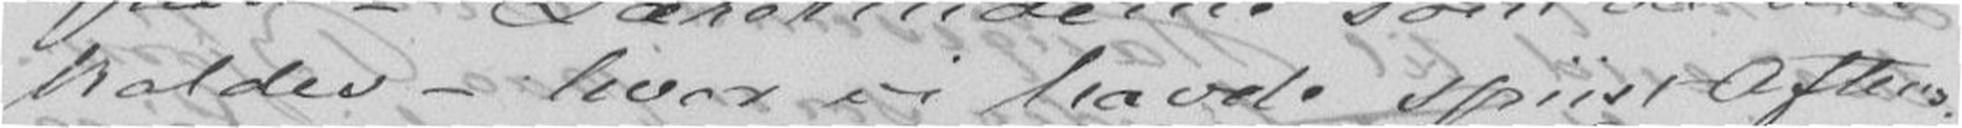holdes - hvor vi havde spiist Aften.
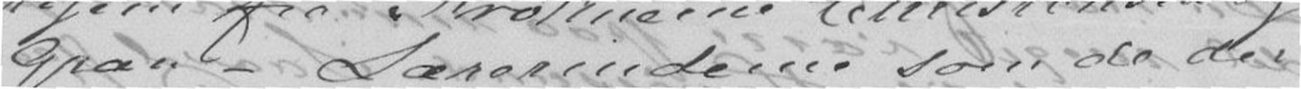Gran - Lærerinderne som de der
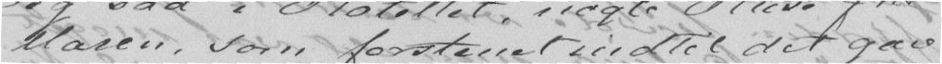Maren, som forstenet indtil det gav
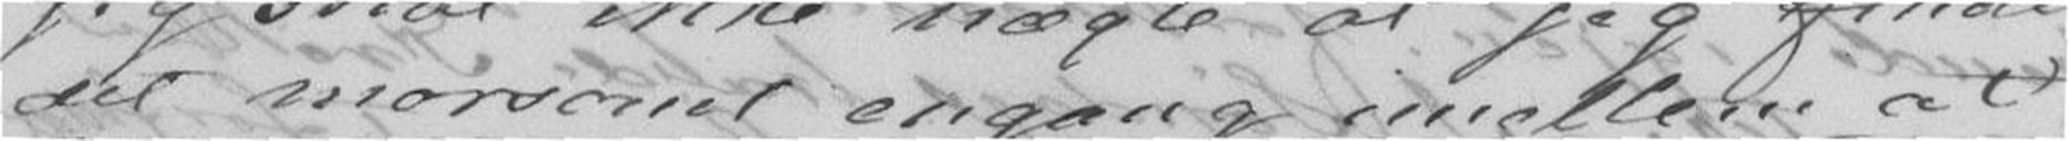det morsomt engang imellem at
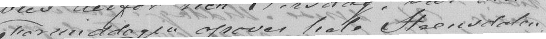Formiddagen opover hele Steinsdalen
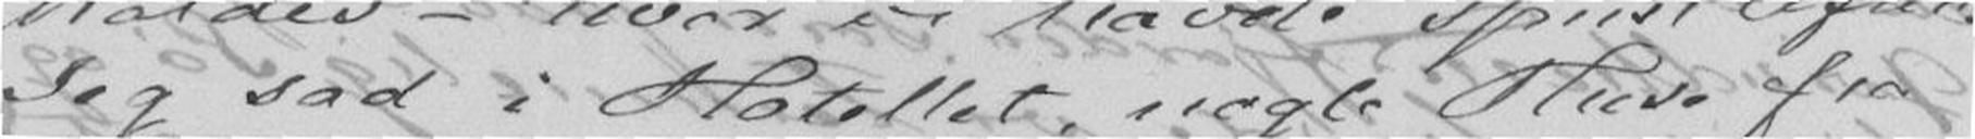Jeg sad i Hotellet, nogle Huse fra
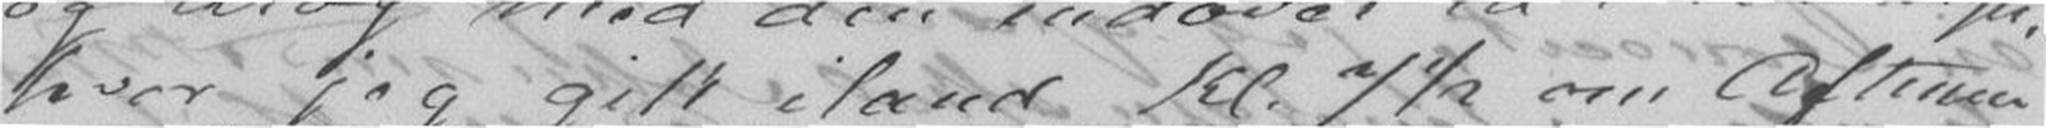hvor jeg gik iland Kl.7½ om Aftenen
